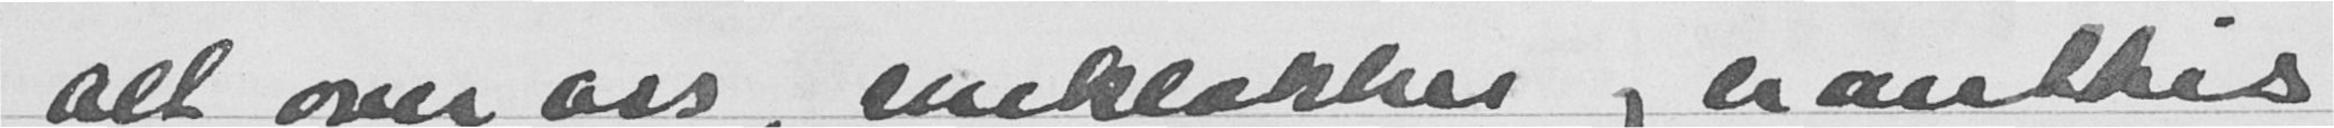alt over oss, sneklokker og erantkes
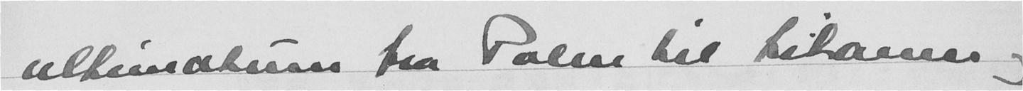ultimatum fra Polen til tilveien og
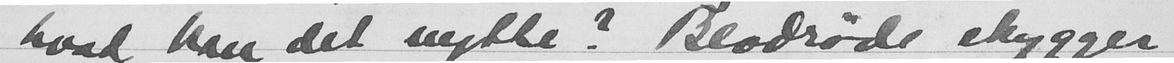hvad kan det nytte? Blodrøde skygger
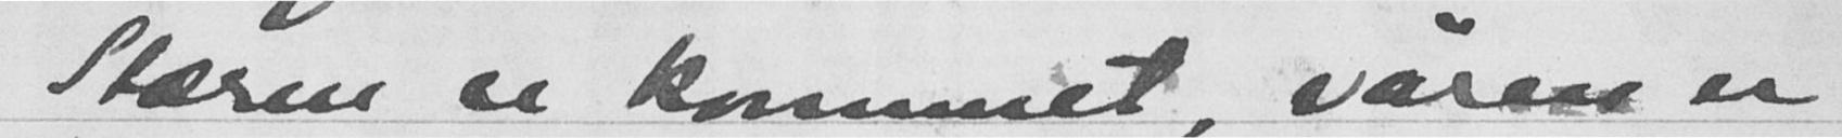Stæren er kommnet, våren er
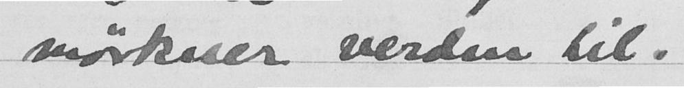mørkner verden til.
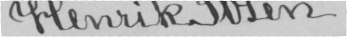Henrik Ibsen.
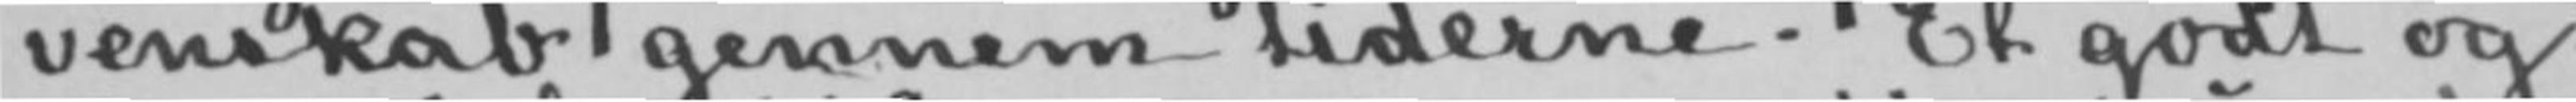venskab gennem tiderne. Et godt og
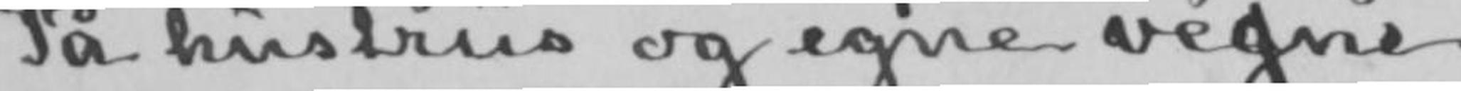På hustrus og egne vegne
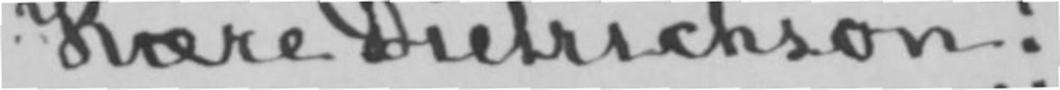Kære Dietrichson!
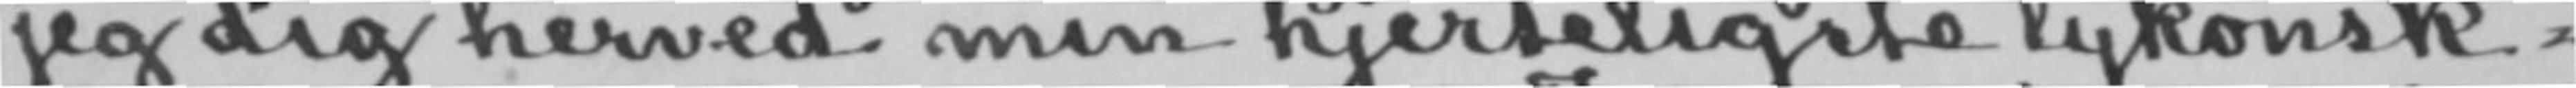jeg dig herved min hjerteligste lykønsk-
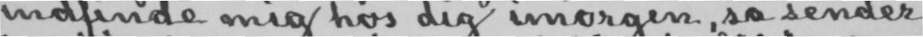indfinde mig hos dig imorgen, så sender
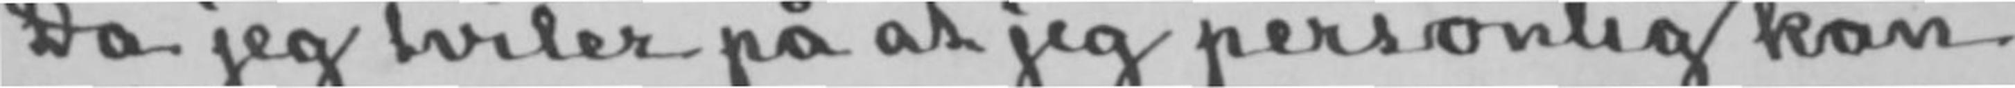Da jeg tviler på at jeg personlig kan
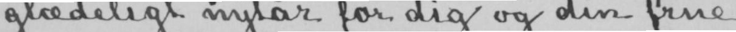glædeligt nytår for dig og din frue!
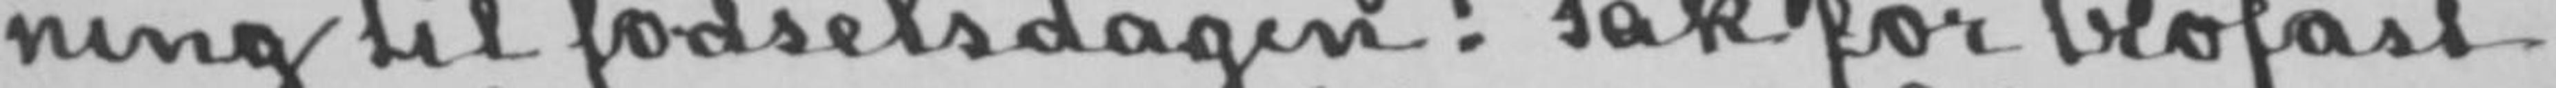ning til fødselsdagen! Tak for trofast
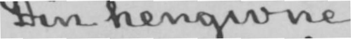Din hengivne
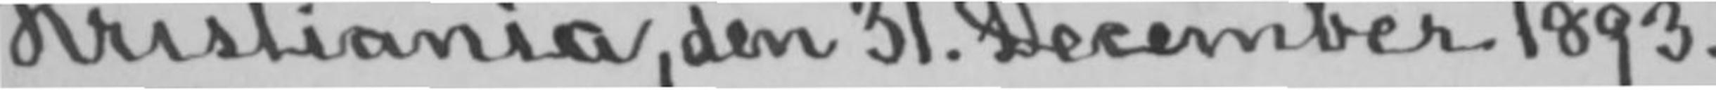Kristiania, den 31. December 1893.
